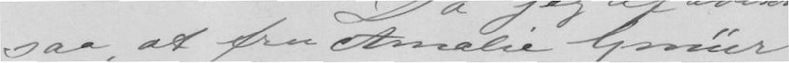saa, at fru Amalie Gmür
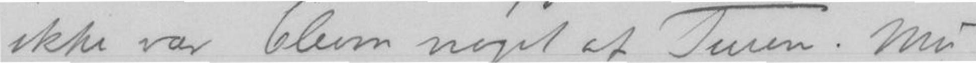ikke var bleven noget af Turen. Min
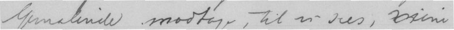Gemalinde modtage, til vi sees, mine
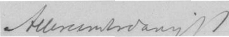Allerunderdanigt
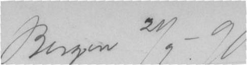Bergen 21/9-90
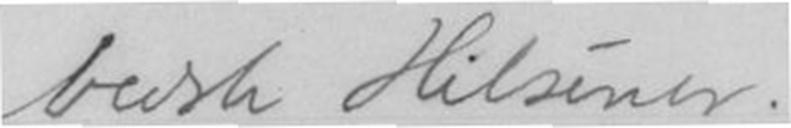bedste Hilsener
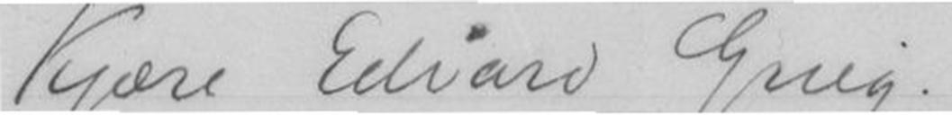Kjære Edvard Grieg.
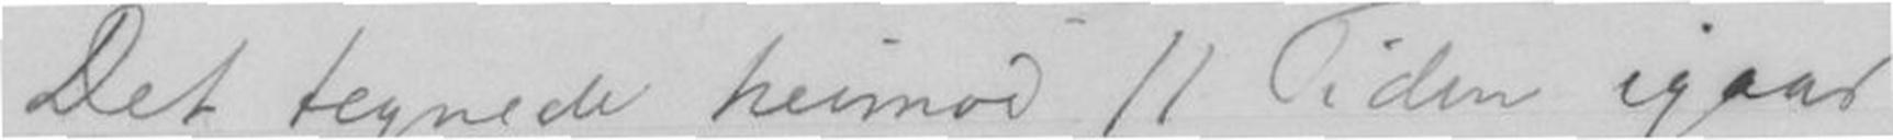Det tegnede heimod 11 Tiden igaar
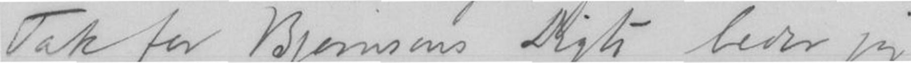Tak for Bjørnsons Digte beder jeg
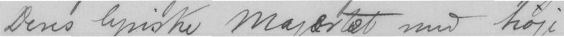Deres lyriske Majestet med høje
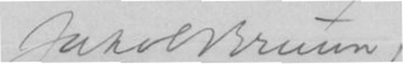Jakob Bruun
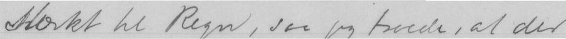Stærkt til Regn, saa jeg troede, at der
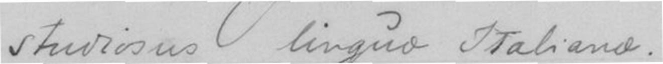studiosus linguo Italiano.
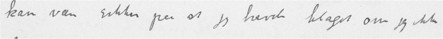kan være sikker paa at jeg havde klaget om jeg ikke
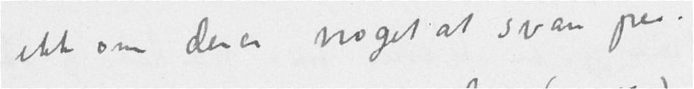ikke om der er noget at svare paa.
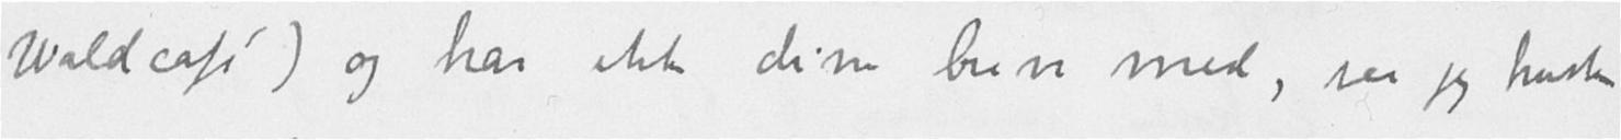Waldcafé) og har ikke dine breve med, saa jeg husker
